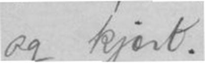og kjært.
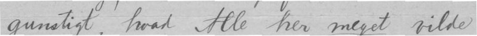gunstigt, hvad Alle her meget vilde
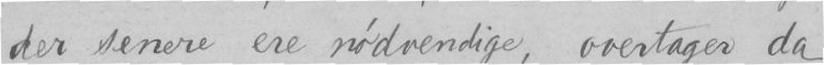der senere ere nødvendige, overtager da
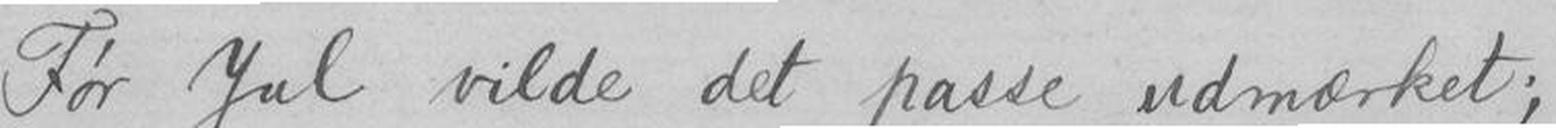Før Jul vilde det passe udmærket;
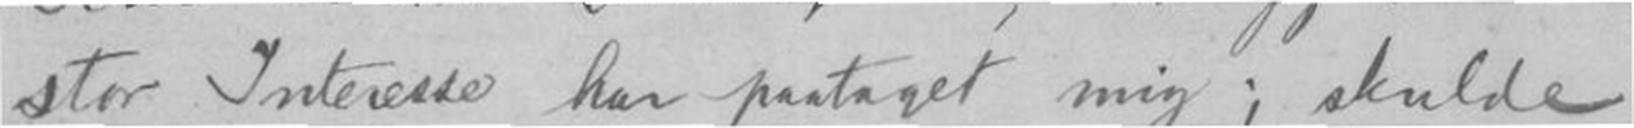stor Interesse har paataget mig; skulde
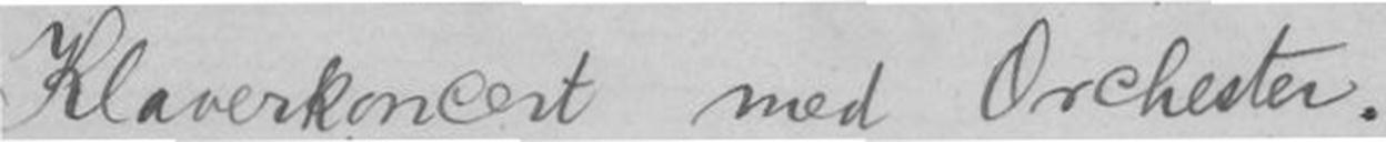Klaverkoncert med Orchester.-
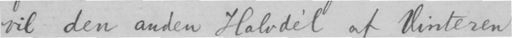vil den anden Halvdél af Vinteren
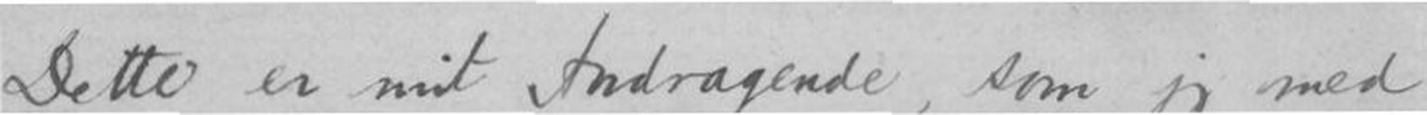Dette er mit Andragende, som jeg med
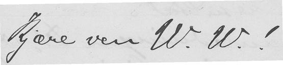Kjære ven W. W.!
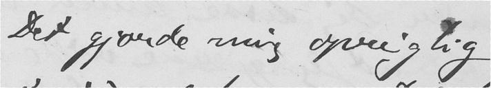Det gjorde mig oprigtig
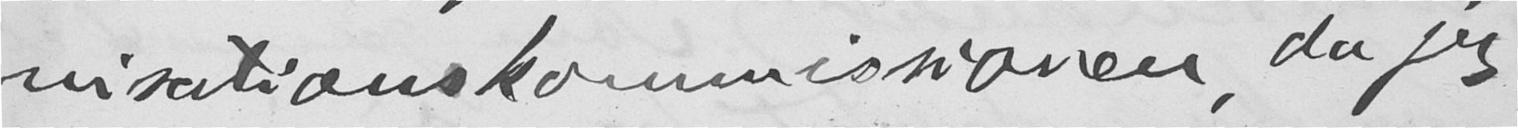nisationskommissionen, da jeg
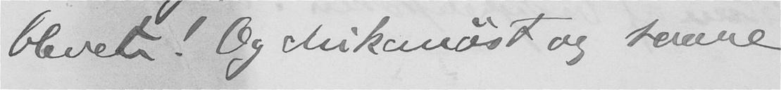blevet! Og chikanøst og saare
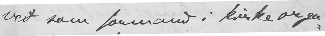vet som formand i kirkeorga-
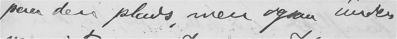paa den plads, men ogsaa under
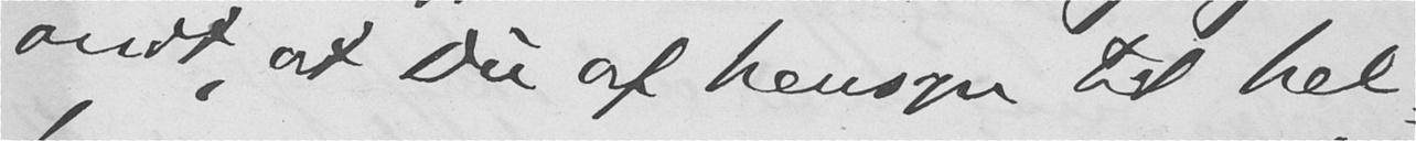ondt, at Du af hensyn til hel-
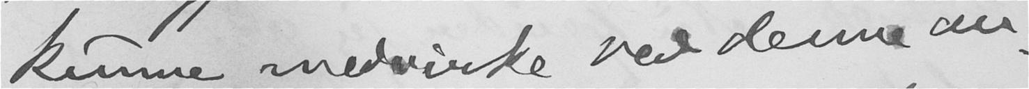kunne medvirke ved denne an-
